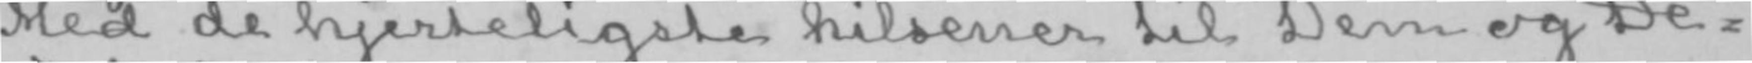Med de hjerteligste hilsener til Dem og De-
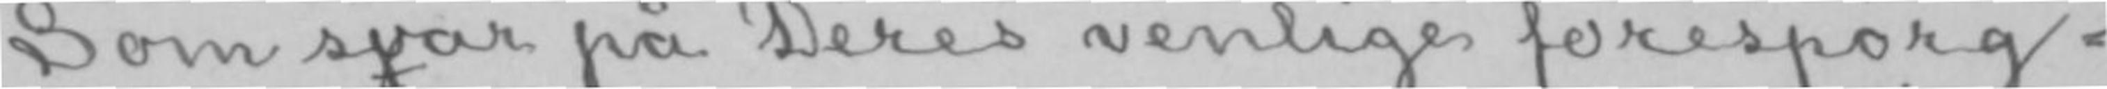Som sjvar på Deres venlige forespørg-
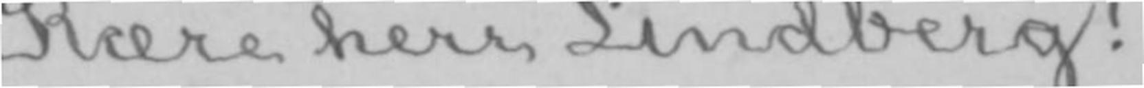Kære herr Lindberg!
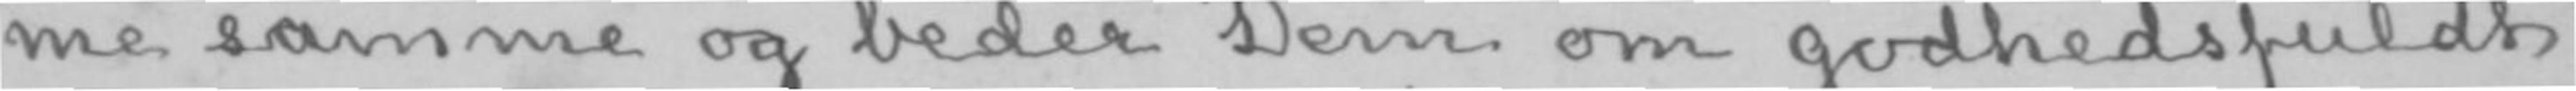me samme og beder Dem om godhedsfuldt
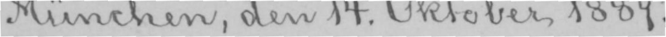München, den 14. Oktober 1889.
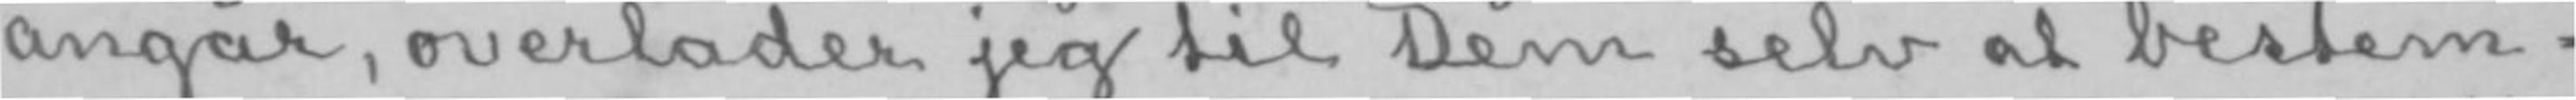angår, overlader jeg til Dem selv at bestem-
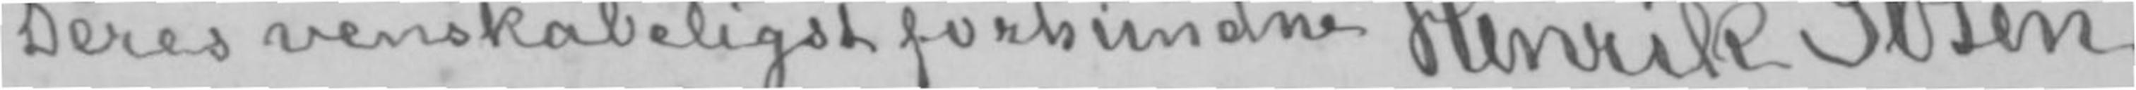Deres venskabeligst forbundne Henrik Ibsen.
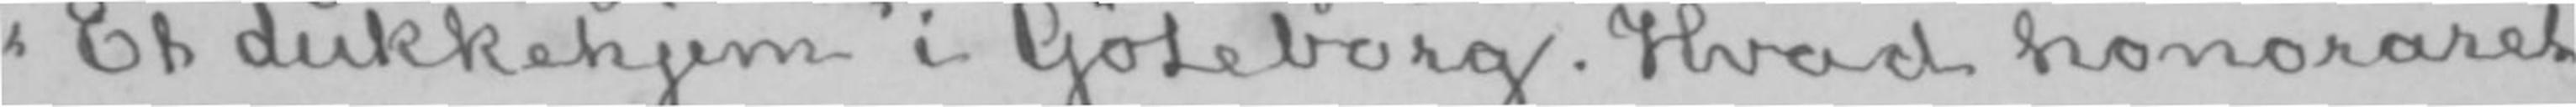«Et dukkehjem» i Göteborg. Hvad honoraret
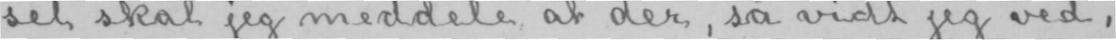sel skal jeg meddele at der, så vidt jeg véd,
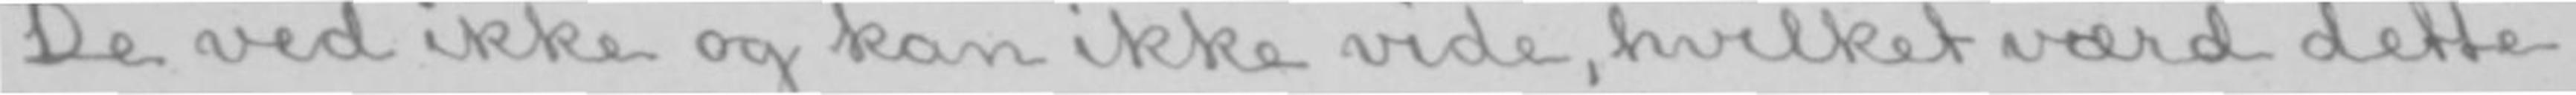De ved ikke og kan ikke vide, hvilket værd dette
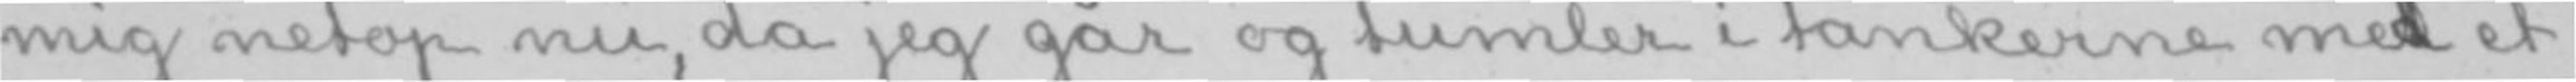mig netop nu, da jeg går og tumler i tankerne med et
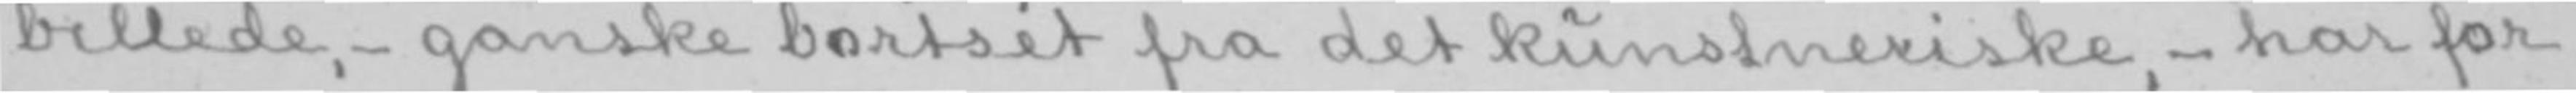billede,- ganske bortsét fra det kunstneriske,- har for
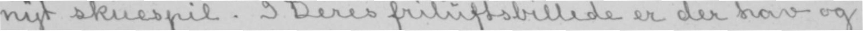nyt skuespil. I Deres friluftsbillede er der hav og
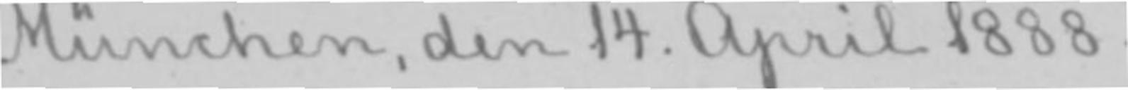München, den 14. April 1888.
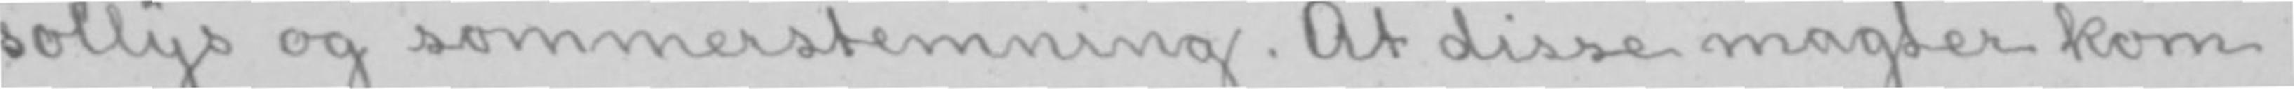sollys og sommerstemning. At disse magter kom
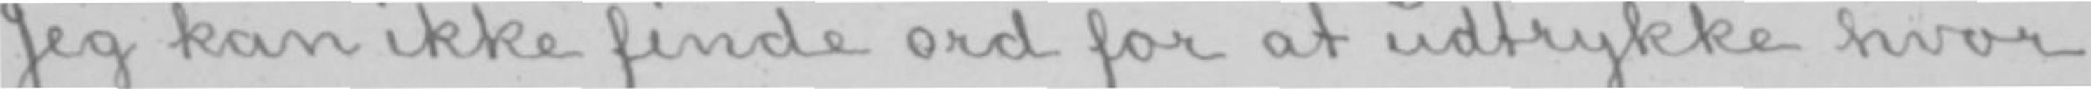Jeg kan ikke finde ord for at udtrykke hvor
